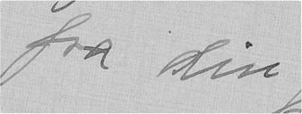fra din
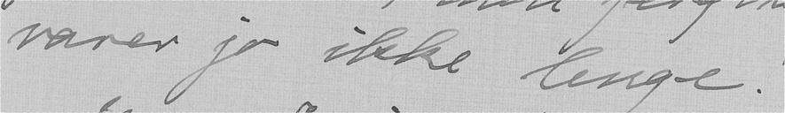varer jo ikke lenge!
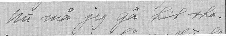Nu må jeg ga til sta-
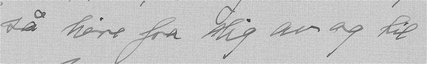så høre fra dig av og til
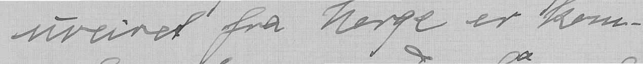uveiret fra Norge er kom-
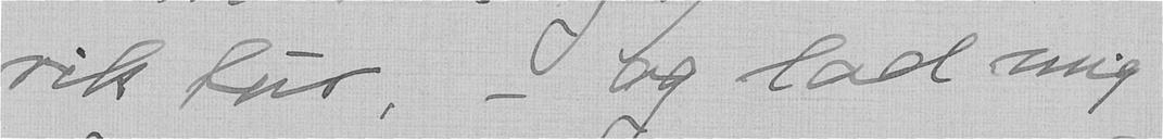rik tur, - og lad mig
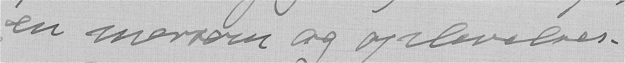en morsom og oplevelses-
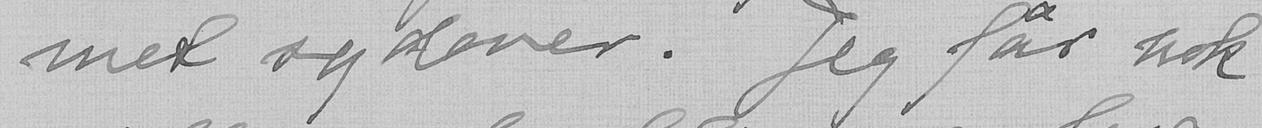met sydover. Jeg får nok
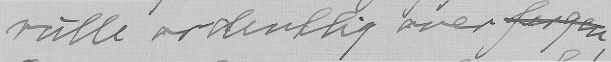rulle ordentlig over fergen
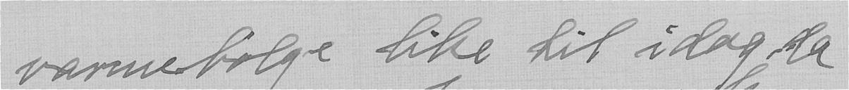varmebølge like til idag, da
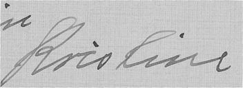Kristine
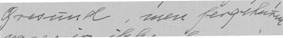Øresund, men fergeturen
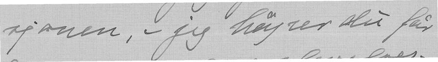sjonen, - jeg håper du får
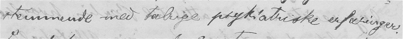stemmende med talrige psykiatriske erfaringer.
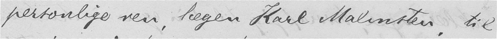personlige ven, lægen Karl Malmsten, til
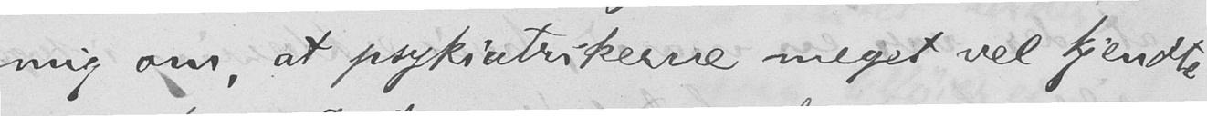mig om, at psykiatrikerne meget vel kjendte
